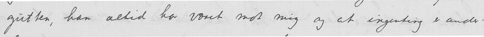gutten, han altid har vært mot mig og at ingenting er ander-
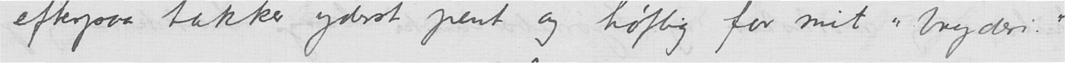efterpaa takker yderst pent og høflig for mit "bryderi".
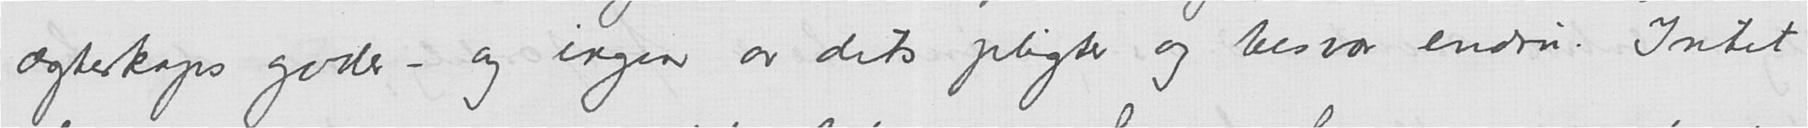ægteskaps goder - og ingen av dets pligter og besvær endnu. Intet
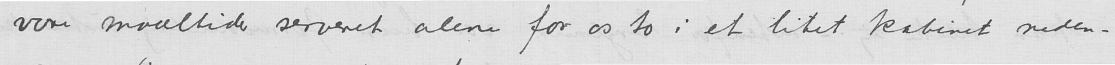vore maaltider servert alene for os to i et litet kabinet neden-
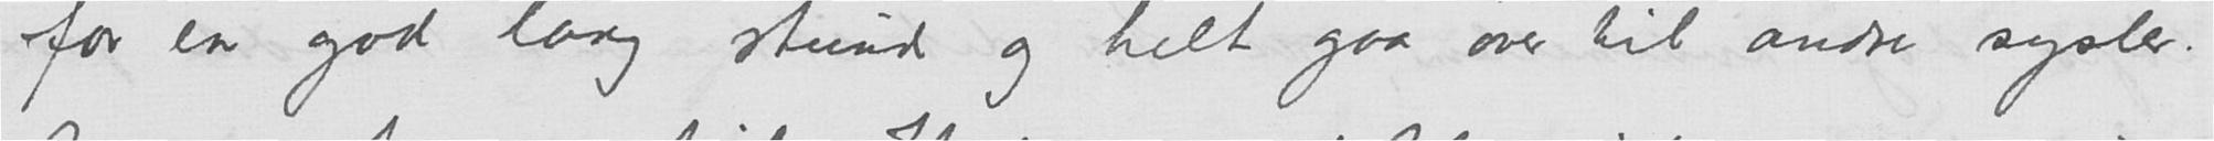for en god lang stund og helt gaa over til andre sysler.
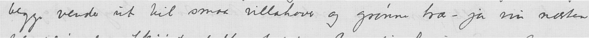begge vender ut til smaa villahaver og grønne trær - ja nu næsten
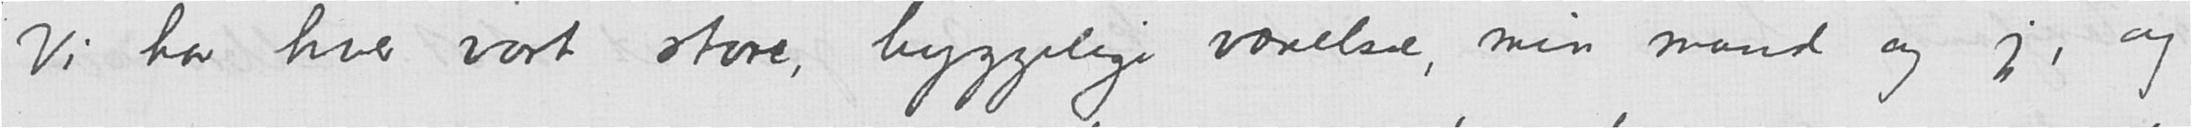Vi har hver vort store, hyggelige værelse, min mand og jeg, og
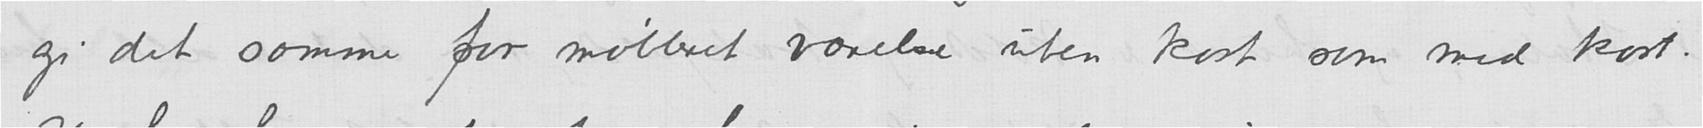gi det samme for møblert værelse uten kost som med kost.
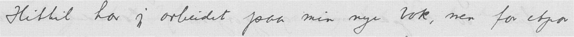Hittil har jeg arbeidet paa min nye bok, men for etpar
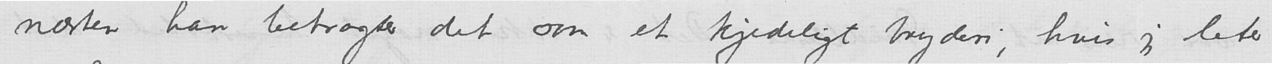næsten han betragter det som et kjedeligt bryderi, hvis jeg leter
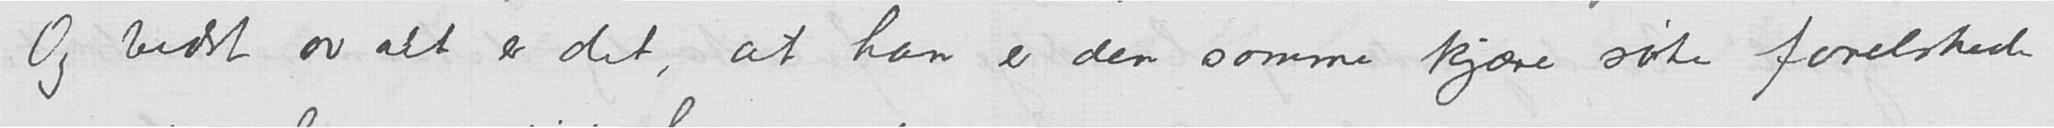Og bedst av alt er det, at han er den samme kjære søte forelskede
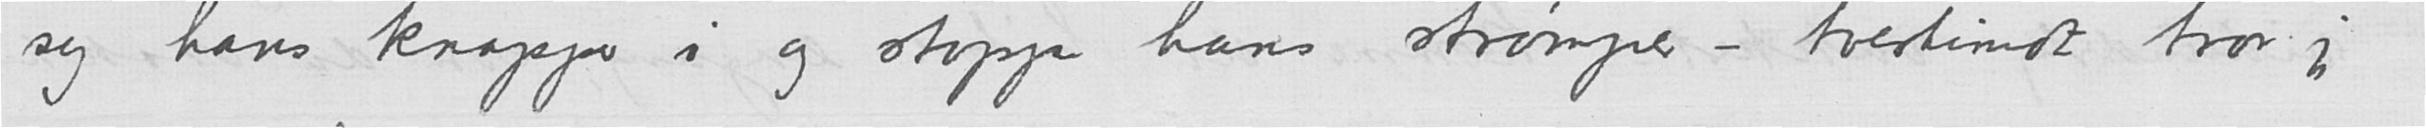sy hans knapper i og stoppe hans strømper - tvertimot tror jeg
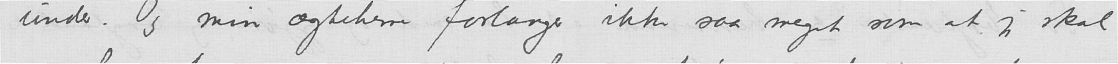under. Og min ægteherre forlanger ikke saa meget som at jeg skal
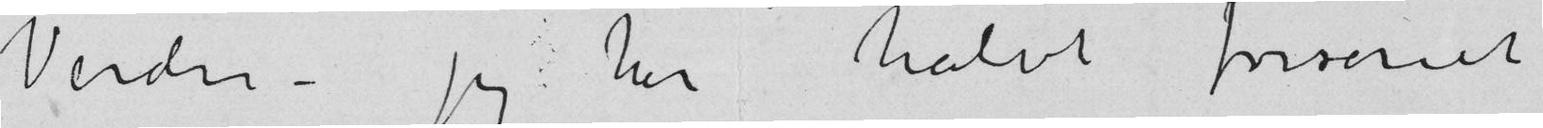Verden – jeg har halvt forsonet
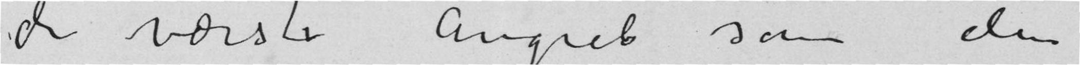de værste Angreb som den
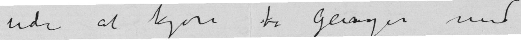ude at kjøre to ganger med
